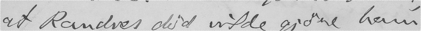at Randves død vilde gjøre ham
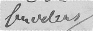broders
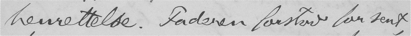henrettelse. Faderen forstod for sent,
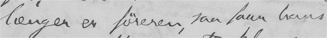længer er føreren, saa faar hans
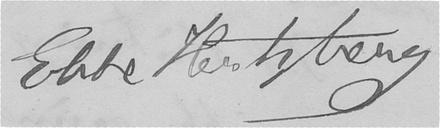Ebbe Hertzberg.
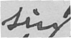sin
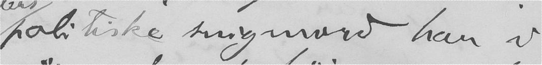politiske snigmord har i
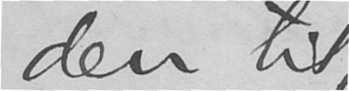den til
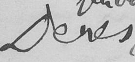Deres
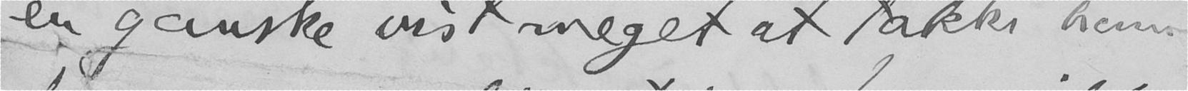er ganske vist meget at takke ham
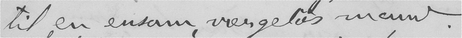til en ensom, værgeløs mand.
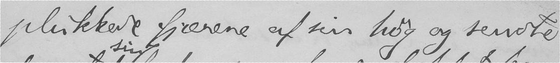plukkede fjærene af sin høg og sendte
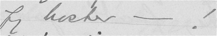Jeg hoster - !
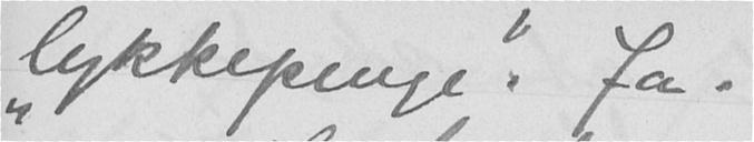"lykkepenge". Ja.
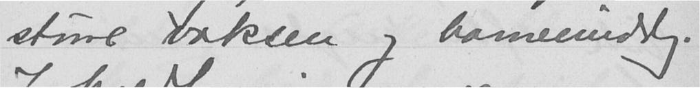større voksen og barnemiddag.
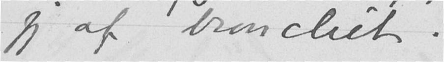jeg af bronchit.
